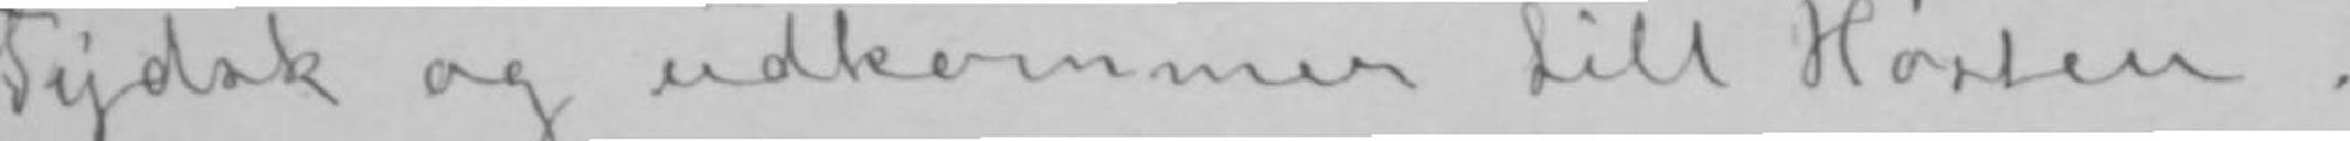Tydsk og udkommer till Høsten.
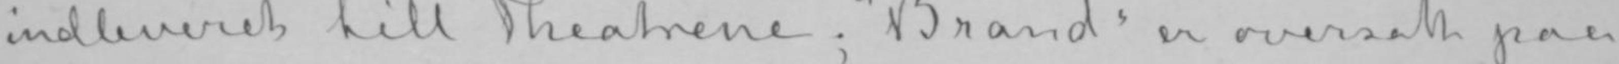indleveret till Theatrene; «Brand» er oversatt paa
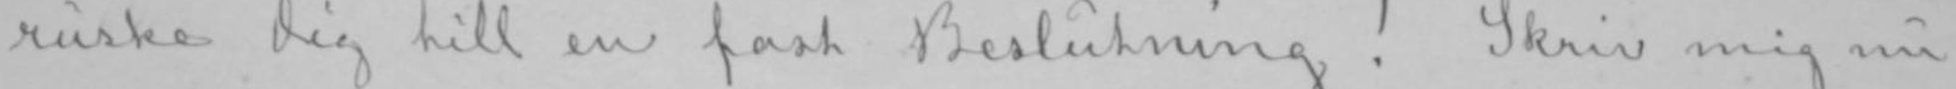ruske Dig till en fast Beslutning! Skriv mig nu
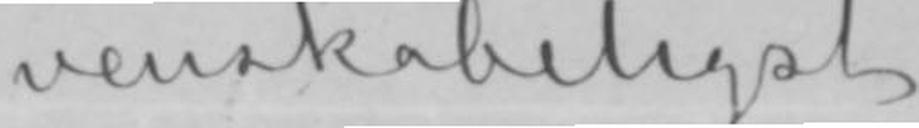venskabeligst
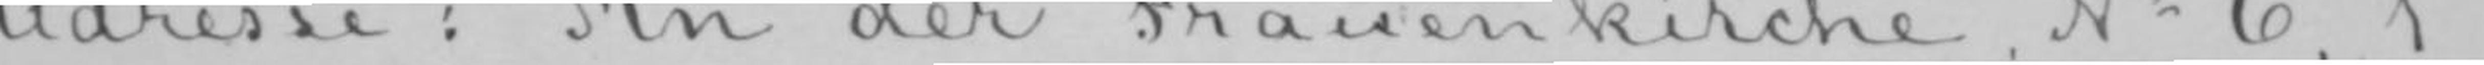Adresse: «An der Frauenkirche, No 6, 1ten
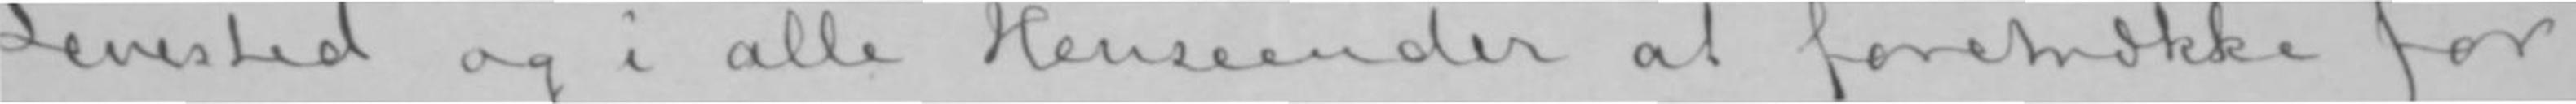Levested og i alle Henseender at foretrække for
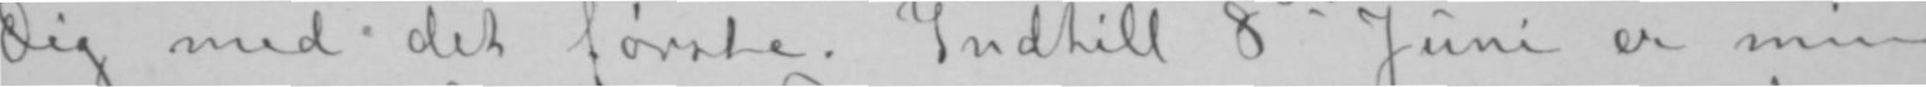Dig med det første. Indtill 8de Juni er min
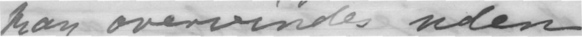kan overvinde uden
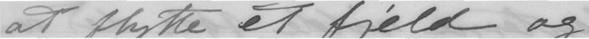at flytte et fjeld og
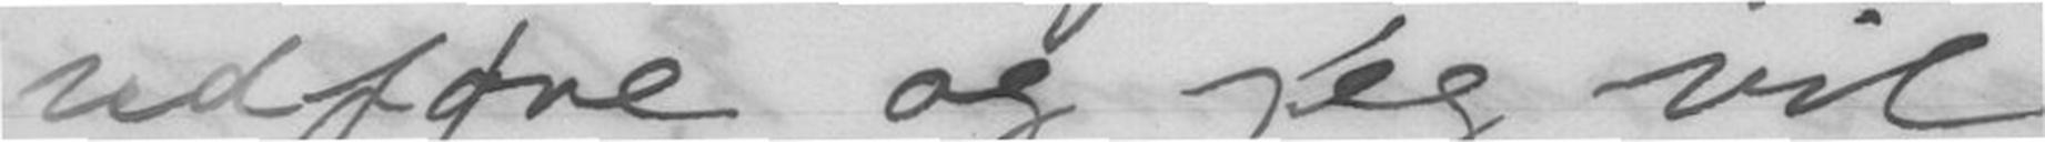udføre og jeg vil
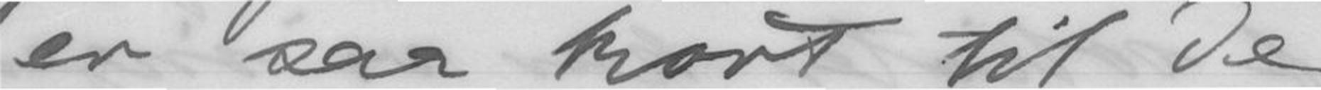er saa kort til De
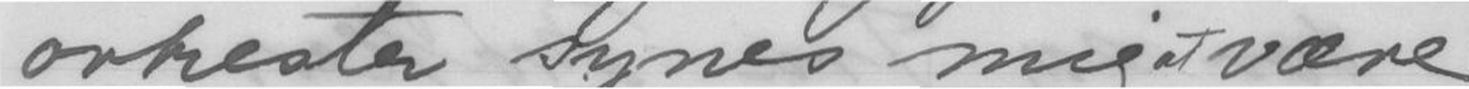orkester synes mig at være
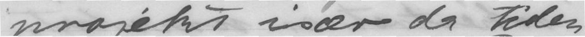prosjekt især da tiden
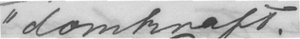"domkraft".
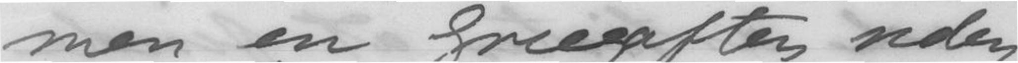men en Griegaften uden
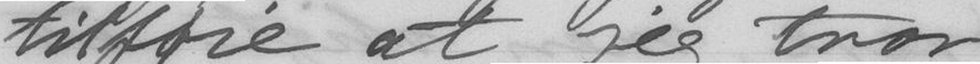tilføre at jeg tror
